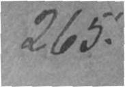265.
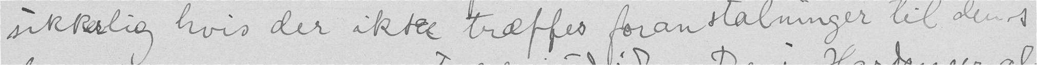sikkerlig hvis der ikke træffes foranstalninger til dens
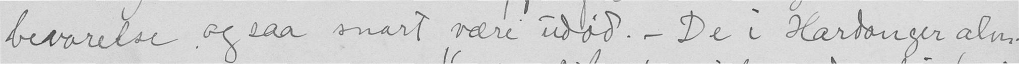bevarelse og saa snart være udød. - De i Hardanger alm.
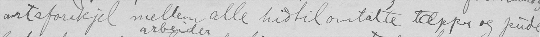artsforskjel mellem alle hidtil omtalte tæpper og pude
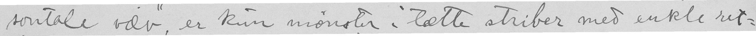sontale væv, er kun mønster i tætte striber med enkle ret-
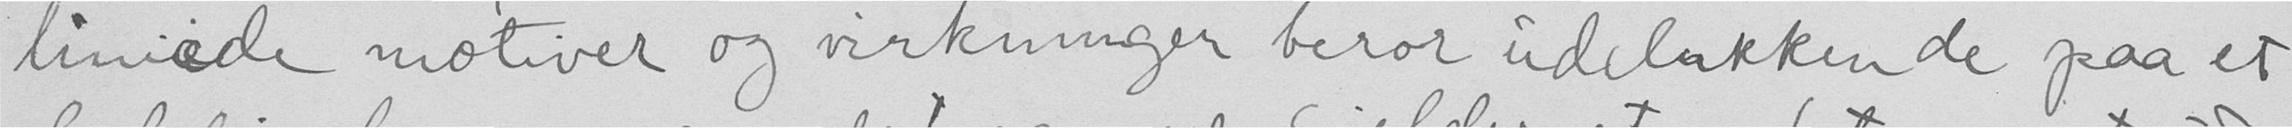liniæde motiver og virkninger beror udelukkende paa et
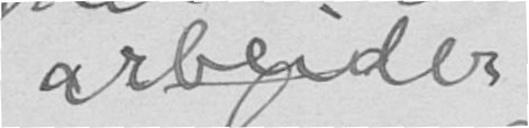arbeider
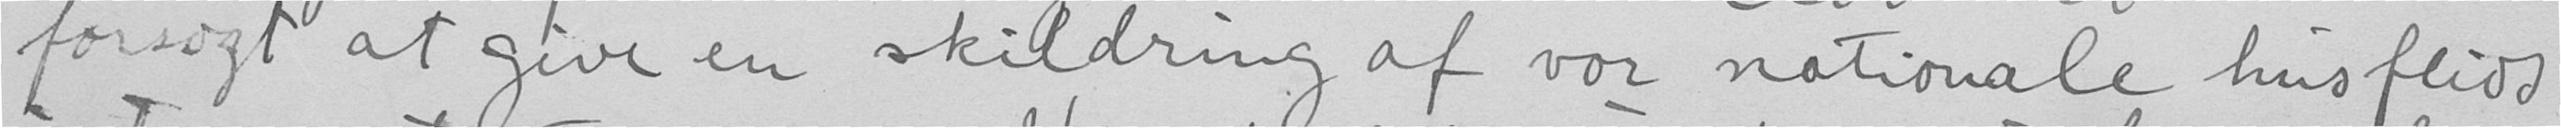forsøgt at give en skildring af vor nationale husflidd
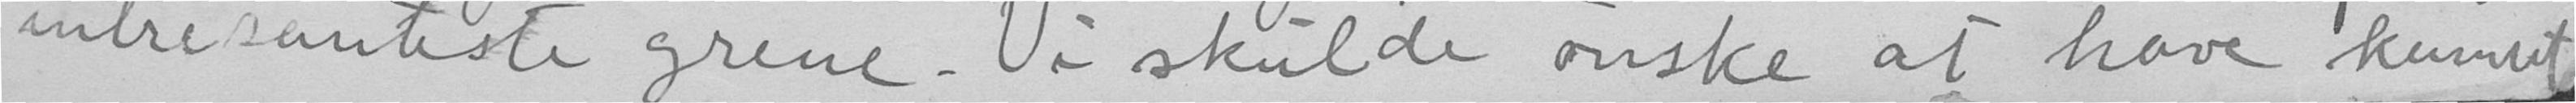intresanteste grene. Vi skulde ønske at have kunnet
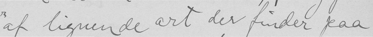af lignende art der finder paa
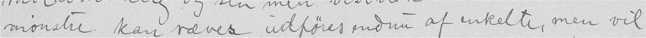mønstre kan væves udføres endnu af enkelte, men vil
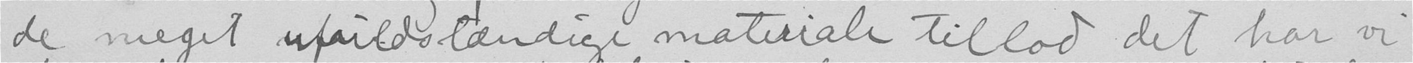de meget ufuldstændige materiale tillod det har vi
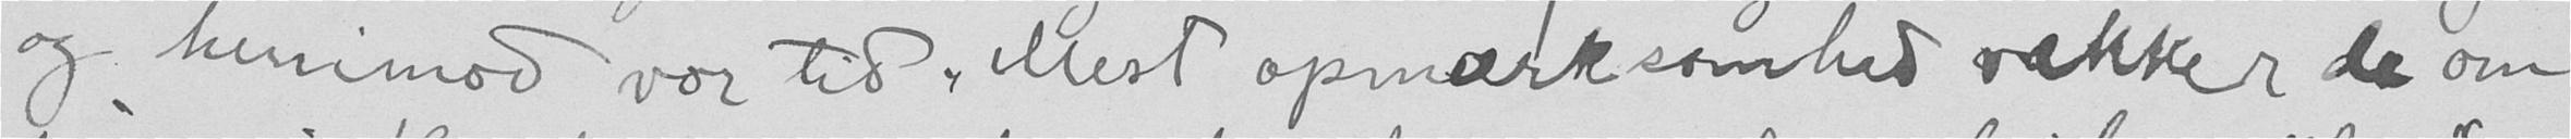og henimod vor tid. Mest opmærksomhed vækker de om
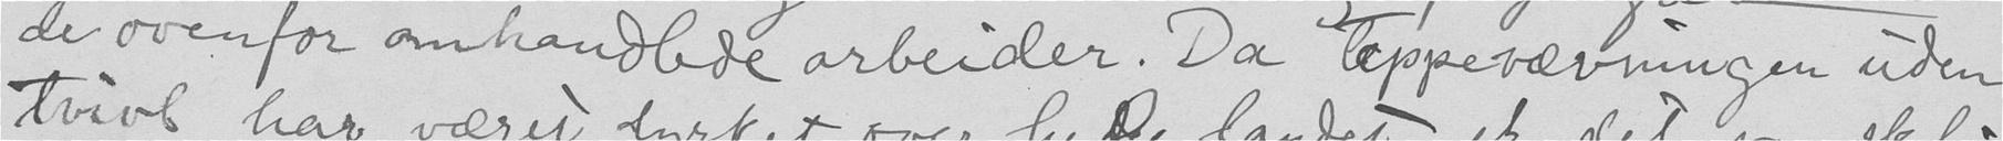de ovenfor omhandlede arbeider. Da tæppevævningen uden
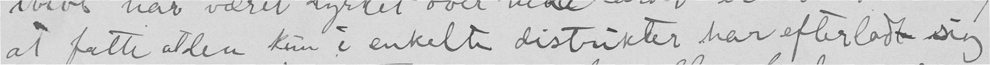at fatte alder kun i enkelte distrikter har efterladt sig
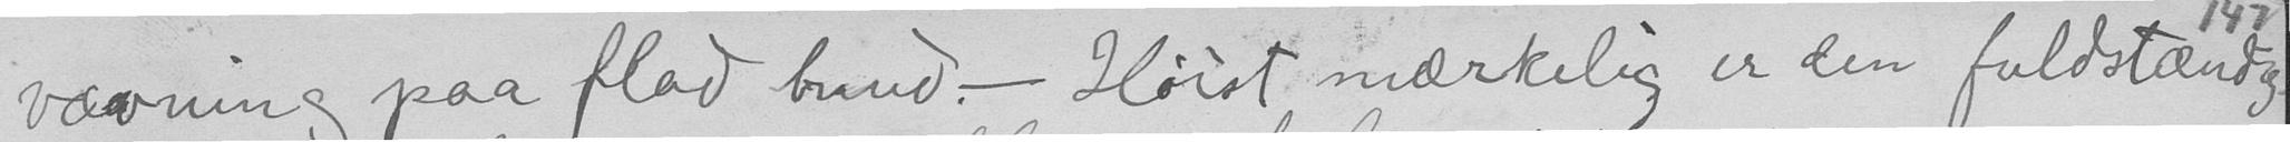vævning paa flad bund,- Høist mærkelig er den fuldstændig
